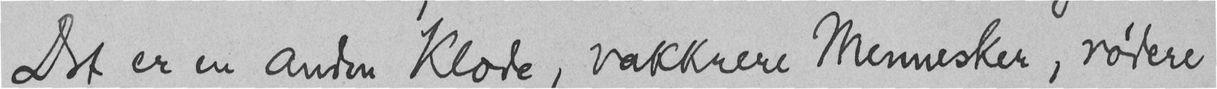Det er en anden Klode, vakkrere Mennesker, rødere
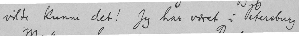vilde kunne det! Jeg har været i Petersburg
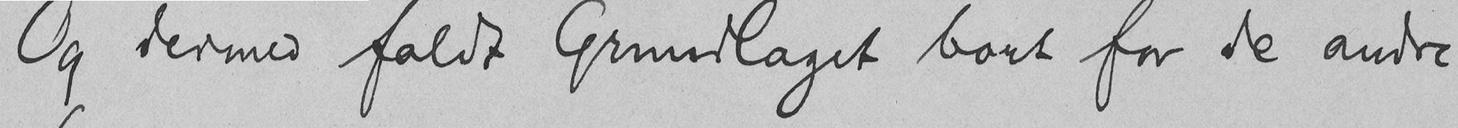Og dermed faldt Grundlaget bort for de andre
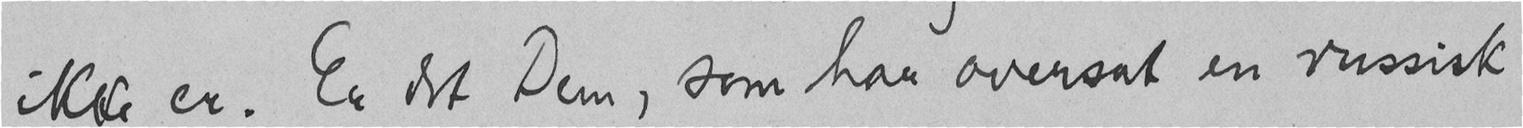ikke er. Er det Dem, som har oversat en russisk
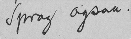Sprog ogsaa.
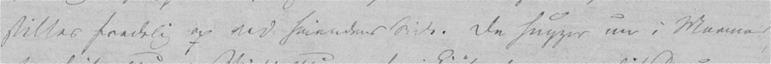stilles fredelig op ved hinandens Side. De hugges nu i Marmor
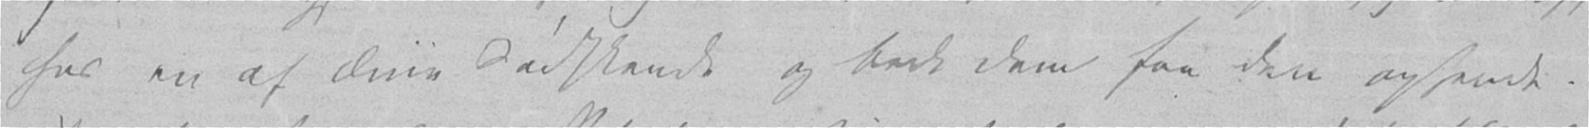hos en af Dine Sødskende og bede dem faa den opsendt.
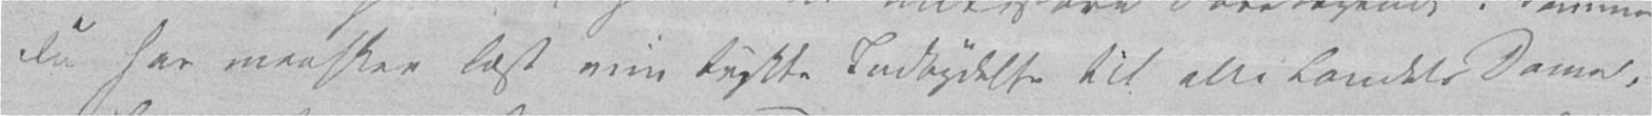Du har maaskee læst min trykte Indbydelse til alle Landets Damer,
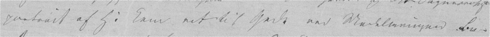portrait af H: kom ret til Gode ved Modelerningen. Bu-
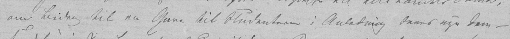om Bidrag til en Gave til Studenterne i Anledning deres nye -
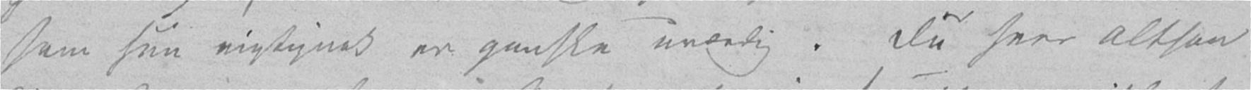som hun rigtignok er ganske uværdig. Du seer altsaa
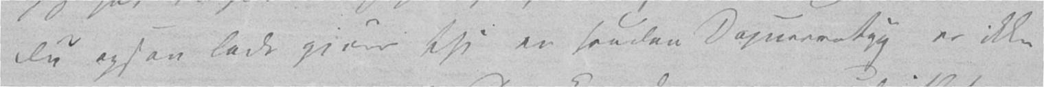Du ogsaa lade gjøre thi en saadan Daguerrotyp er ikke
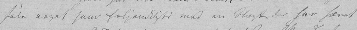føle noget som Erkjendtlighed mod en Slægt der har hævet
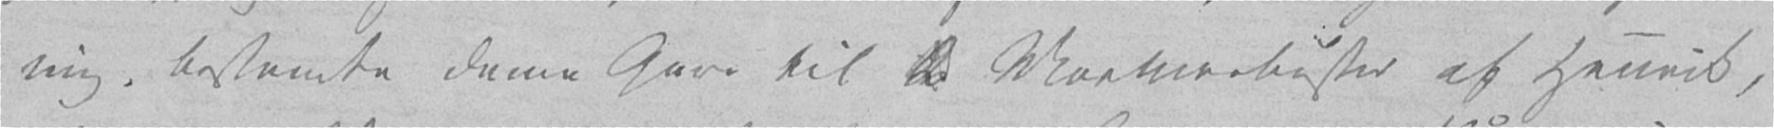mig, bestemte denne Gave til 2 Marmorbuster af Henrik,
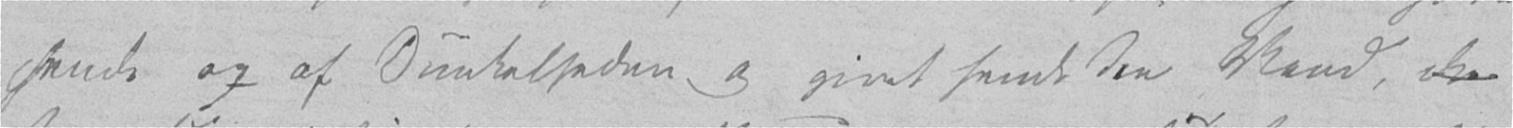hende op af Dunkelheden og givet hende den Mand, der
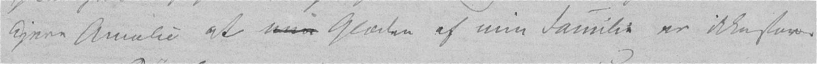kjere Amalie at min Glæden af min Familie er ikke stor.
